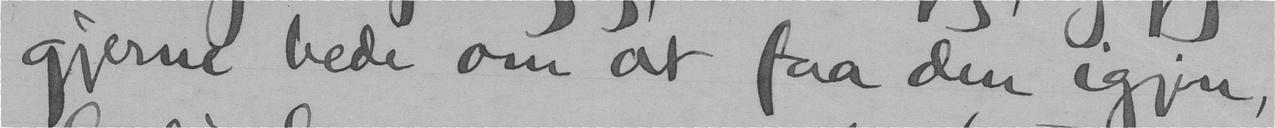gjerne bede om at faa dem igjen,
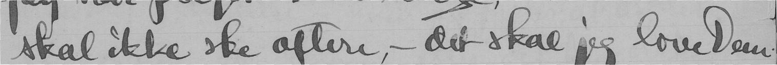skal ikke ske oftere - det skal jeg love Dem.
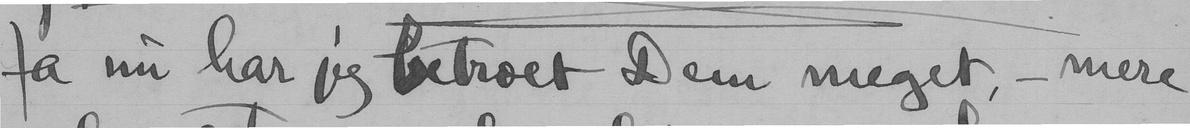Ja nu har jeg betroet Dem meget - mere
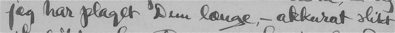jeg har plaget Dem længe, - akkurat slikt
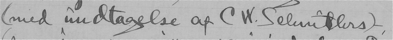(med undtagelse af CW. Selmtlers),
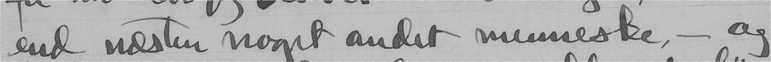end næsten noget andet menneske, - og
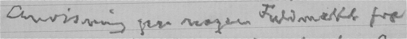Anvisning paa nogen Fuldmakt fra
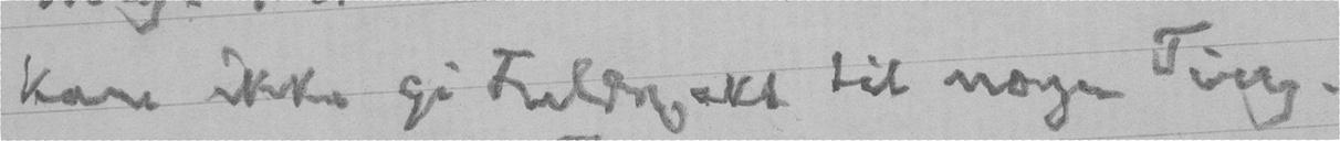kan ikke gi Fuldmakt til nogen Ting.
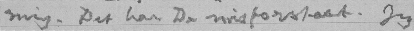mig. Det har De misforstaat. Jeg
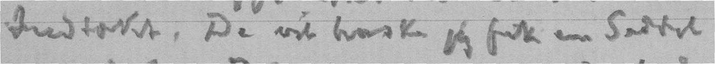Indtækt. De vil huske jeg fik en Seddel
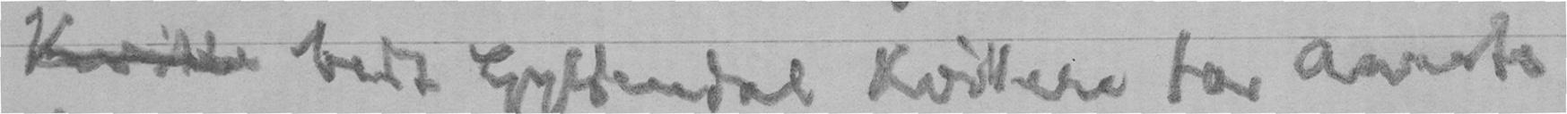kvitte bedt Gyldendal kvittere for Aarets
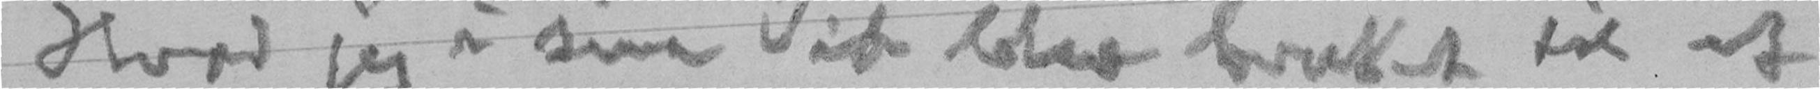Hvad jeg i sin Tid blev brukt til et
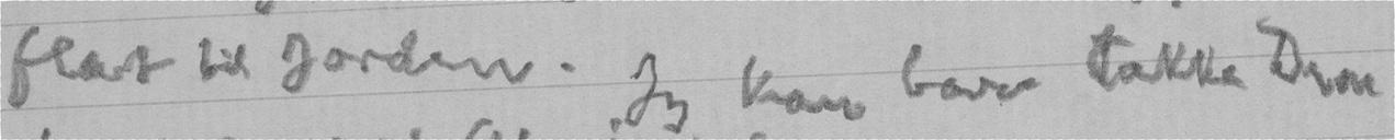flat til Jorden. Jeg kan bare takke Dem
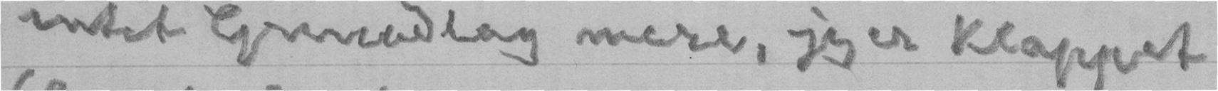intet Grundlag mere, jeg er klappet
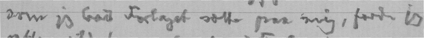som jeg bad Forlaget sætte paa mig, fordi jeg
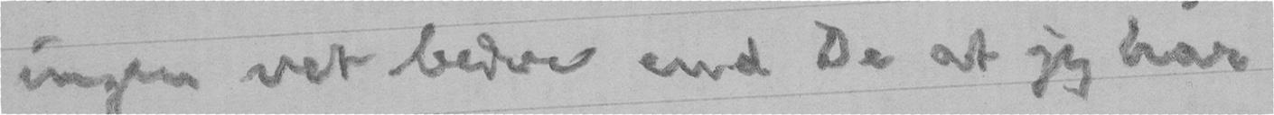ingen vet bedre end De at jeg har
